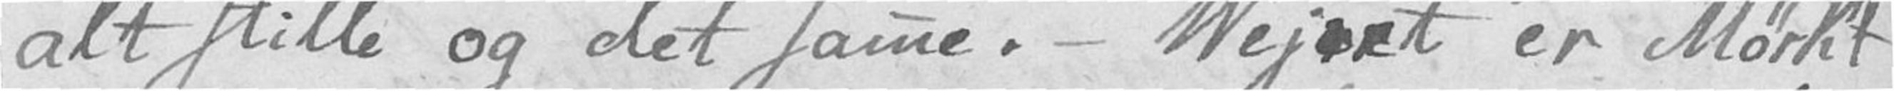alt stille og det samme. – Vejret er Mørkt
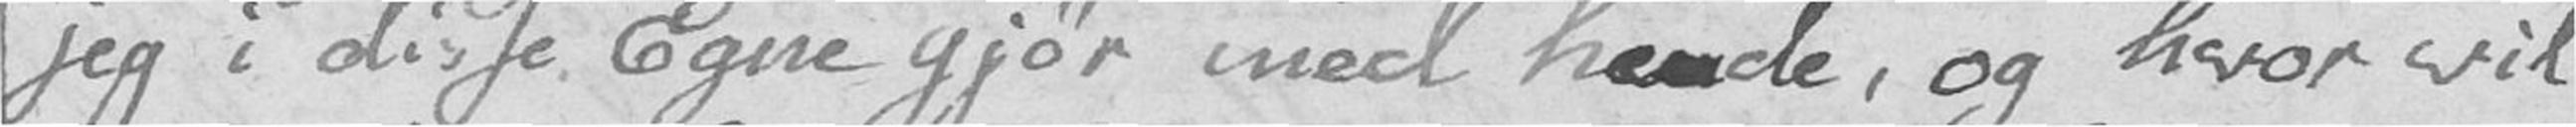jeg i disse Egne gjør med hende, og hvor vil
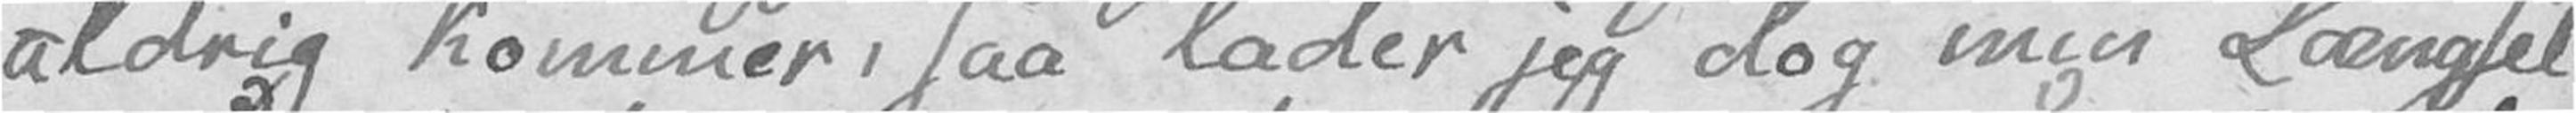aldrig kommer, saa lader jeg dog min Længsel
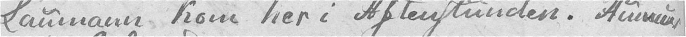Laumann kom her i Aftenstunden. Humeu
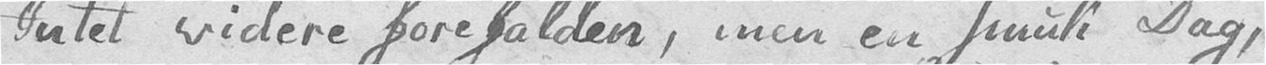Intet videre forefalden, men en smuk Dag,
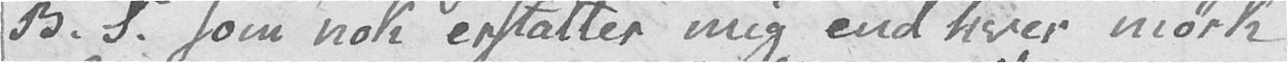B. S. som nok erstatter mig end hver mørk
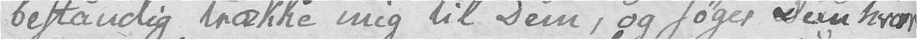bestandig trække mig til Dem, og søger Dem hvor
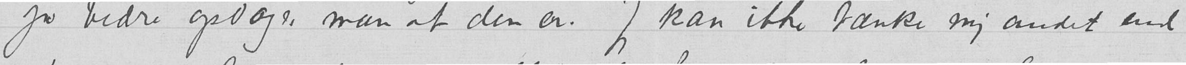jo bedre opdager man at den er. Jeg kan ikke tænke mig andet end
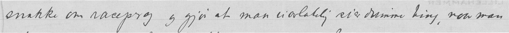snakke om racepræg og gjør at man uavlatelig sier dumme ting, naar man
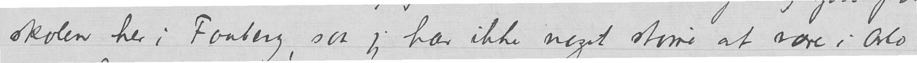skolen her i Faaberg, saa jeg har ikke noget større at være i Oslo
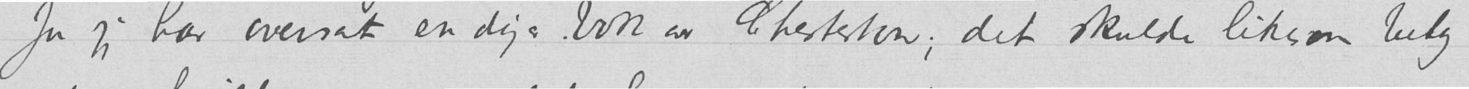Ja jeg har oversat en diger bok av Chesterton; det skulde likesom bety
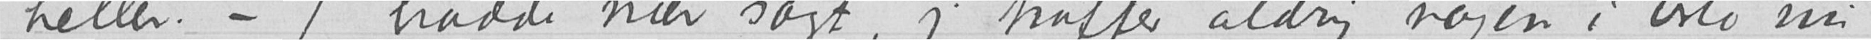heller – Jeg hadde nær sagt, jeg træffer aldrig nogen i Oslo nu,
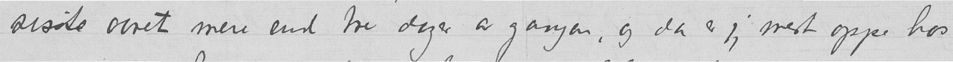sisste aaret mere end tre dager av gangen, og da er jeg mest oppe hos
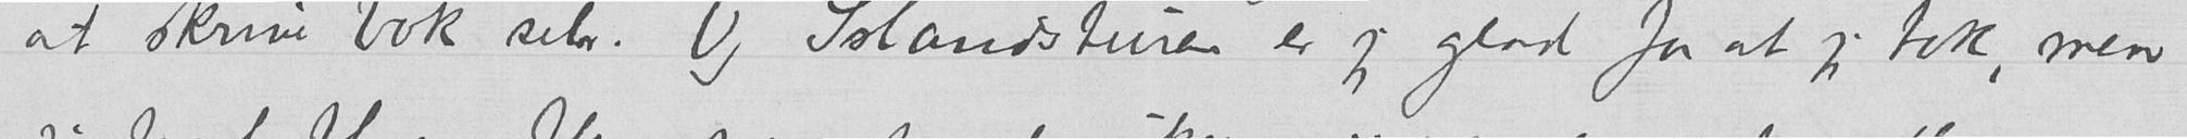at skrive bok selv. Og Islandsturen er jeg glad for at jeg tok, men
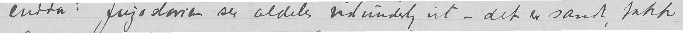endda? Jugeslavien ser aldeles vidunderlig ut – det er sandt, takk
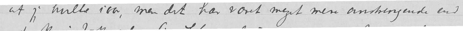at jeg hvilte iaar, men det har været meget mere anstrengende end
